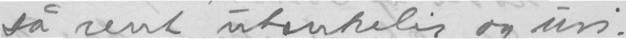så rent utænkelig og uri-
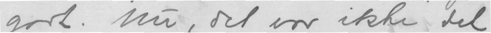godt. Nu, det var ikke det
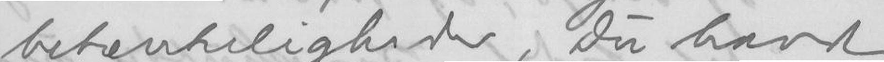betænkeligheder, Du havde
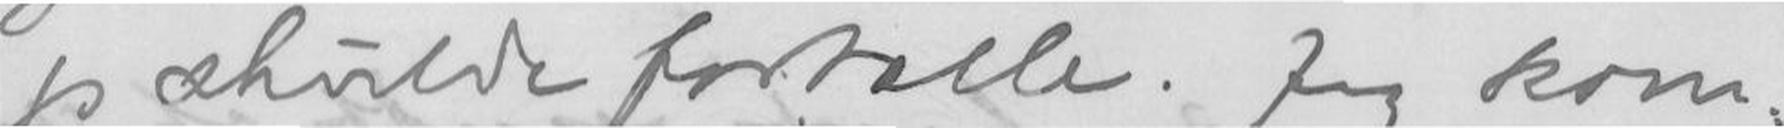jeg skulde fortælle. Jeg kom-
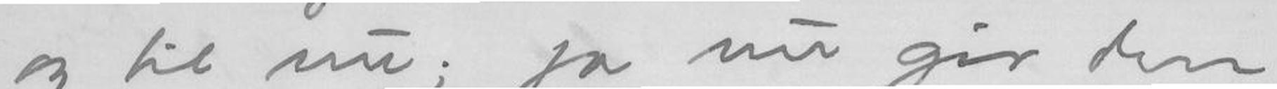og til nu; ja nu gir den
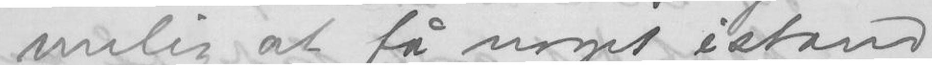melig at få noget istand
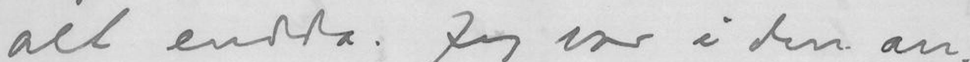alt endda. Jeg var i den an-
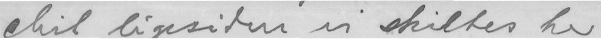chit ligesiden vi skiltes her
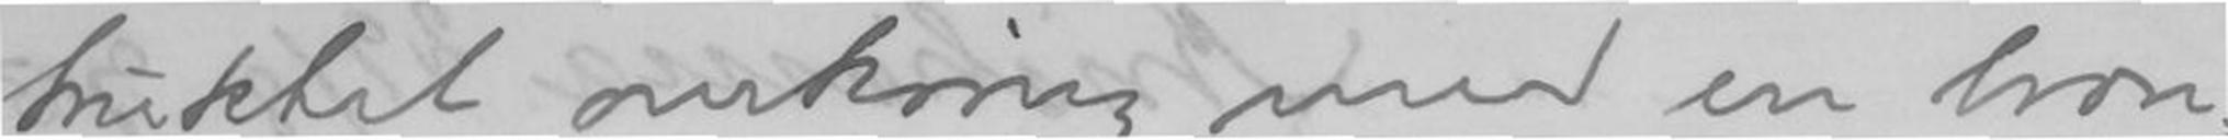truktet omkring med en bron-
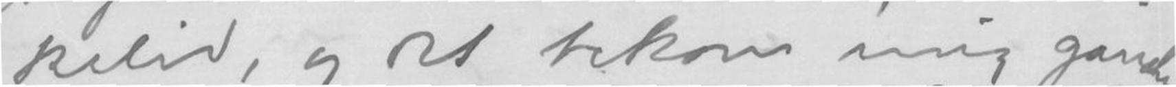kelid, og det bekom mig ganske
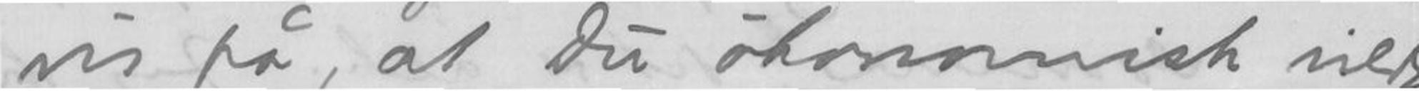vis på, at Du økonomisk vilde
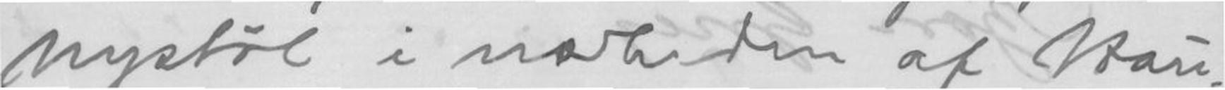Nystøl i nærheden af Hau-
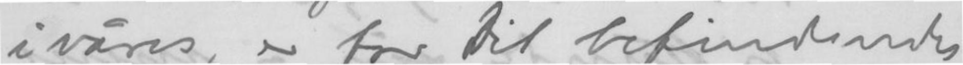ivåres, er for Dit befindendes
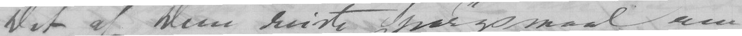Det af Dem reiste spørgsmaal om
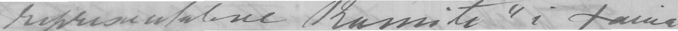representabne Komite i Xania
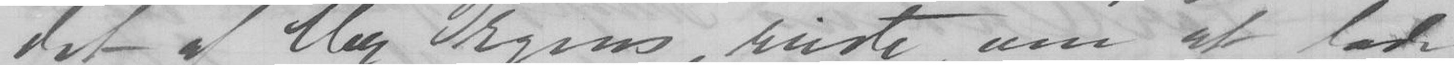det af Her Irgens reiste, om at lade
